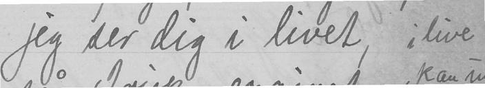jeg ser dig i livet, i live
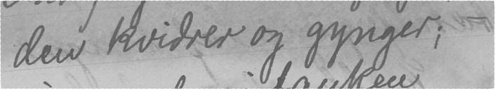den kvidrer og gynger; -
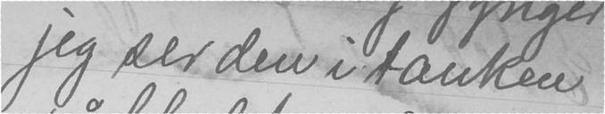jeg ser den i tanken
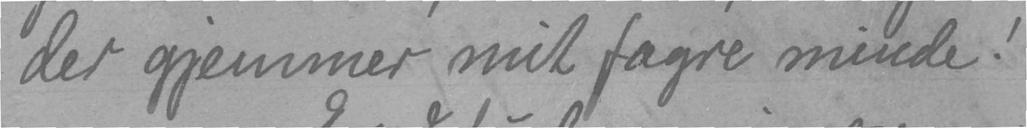der gjemmer mit fagre minde!
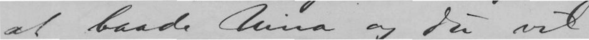at baade Nina og Du vil
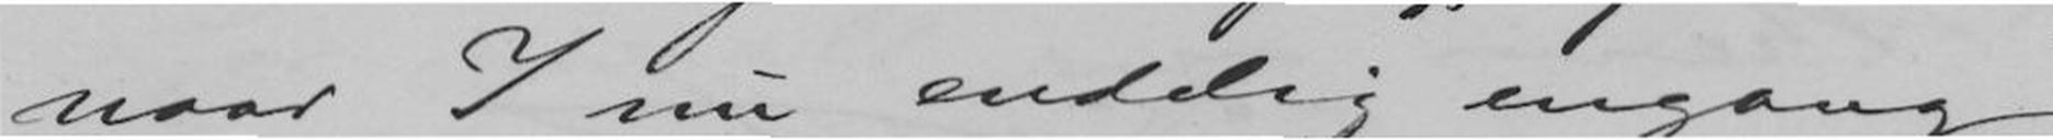naar I nu endelig engang
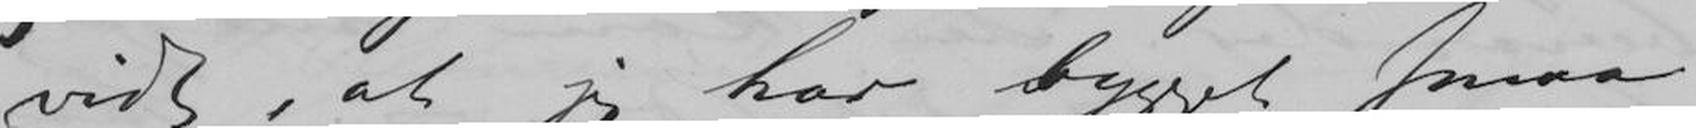vidt, at jeg har bygget smaa
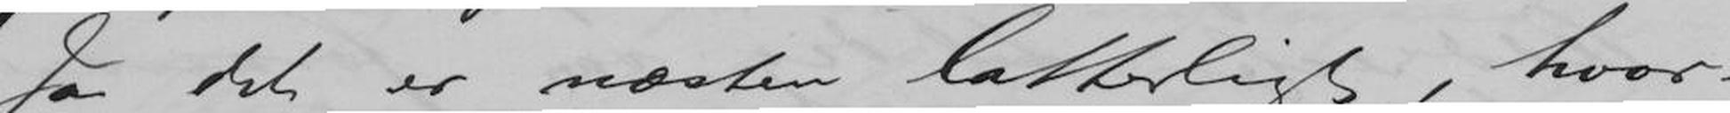Ja det er næsten latterligt, hvor-
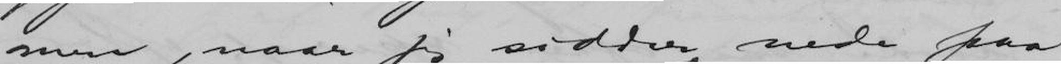men, naar jeg sidder nede paa
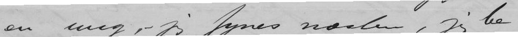en ung, - jeg synes næsten, jeg be-
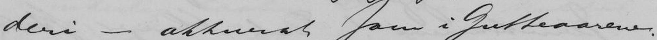deri - akkurat som i Gutteaarene.
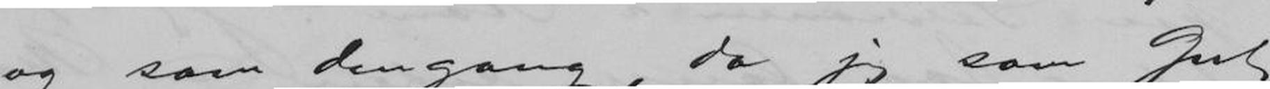og som dengang, da jeg som Gut
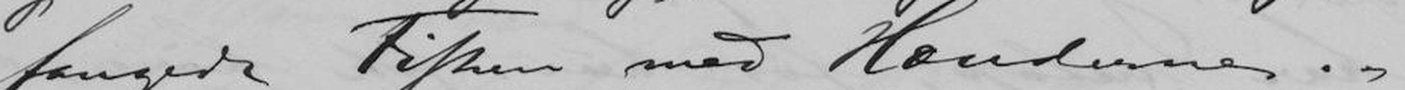fangede Fisken med Hænderne.
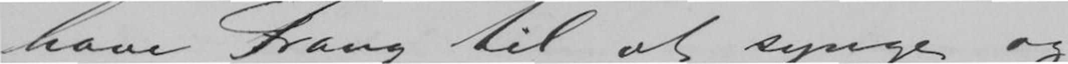have Trang til at synge og
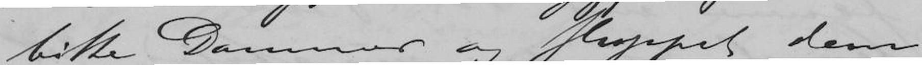bitte Dammer og sluppet dem
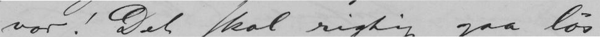vor! Det skal rigtig gaa løs,
